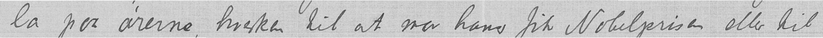la paa ørerne hverken til at mor hans fik Nobelprisen eller til
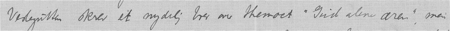Veslegutten skrev et nydelig brev over themaet "Gud alene æren", men
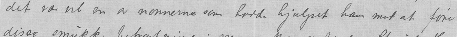det var vel en av nonnerne som hadde hjulpet ham med at føre
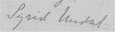Sigrid Undset.
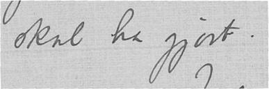skal ha gjort.
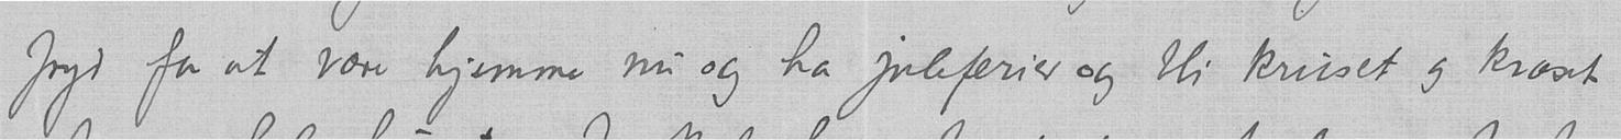fryd for at være hjemme nu og ha juleferie og bli kruset og kræset
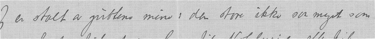Jeg er stolt av guttene mine : den store ikke saa meget som
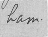ham.
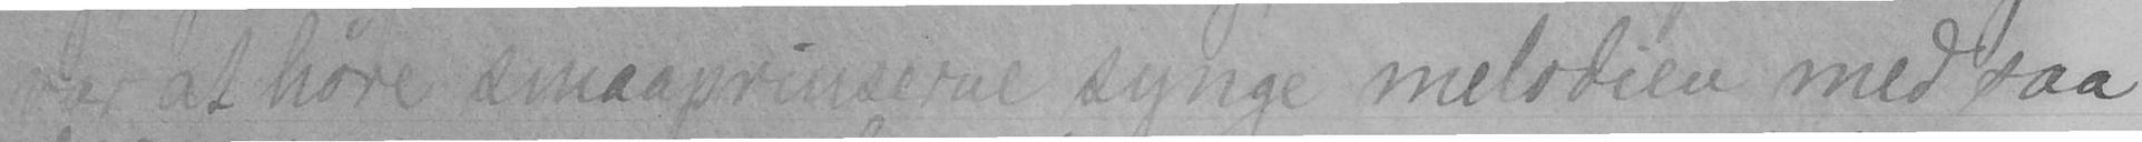var at høre smaaprinserne synge melodien med saa
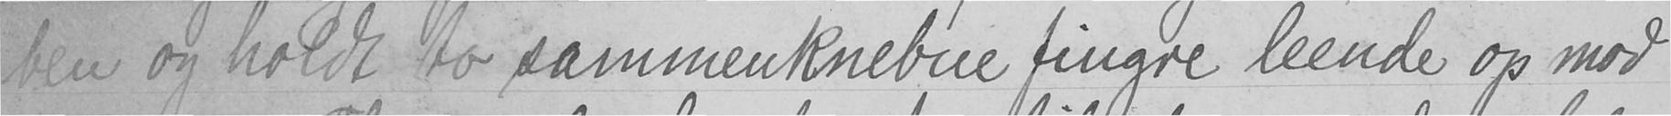ben og holdt to sammenknebne fingre leende op mod
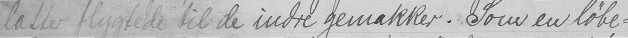latter flygtede til de indre gemakker. Som en løbe-
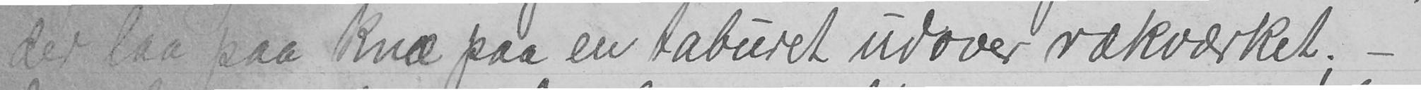der laa paa knæ paa en taburet udover rækværket; -
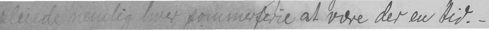pleide nemlig hver sommerferie at være der en tid. -
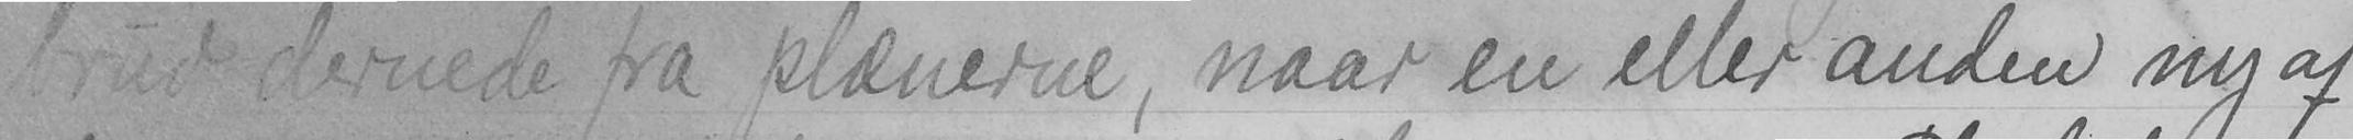brud dernede fra plænerne, naar en eller anden ny af
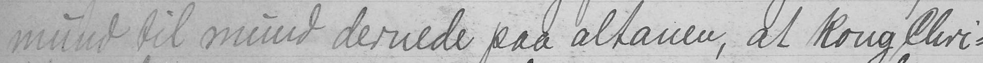mund til mund dernede paa altanen, at Kong Chri-
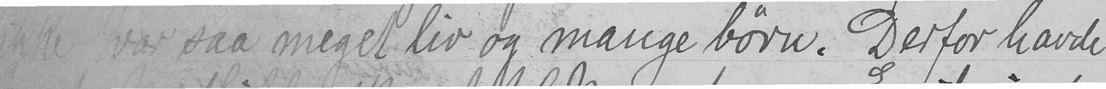var saa meget liv og mange børn. Derfor havde
